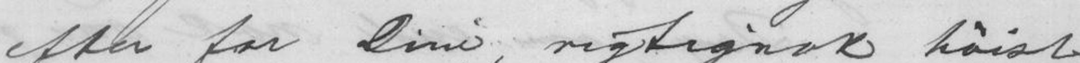efter for Dine, rigtignok høist
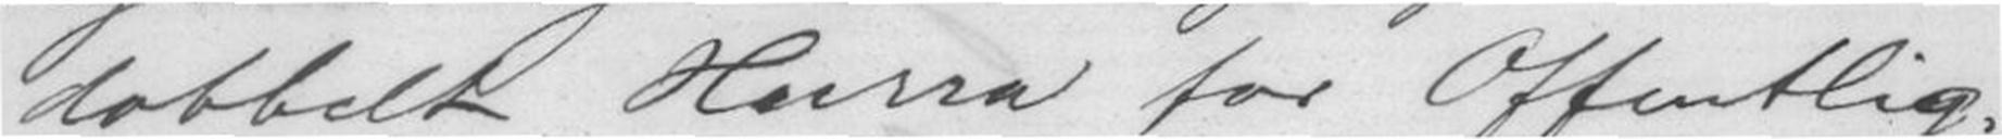dobbelt Hurra for Offentlig-
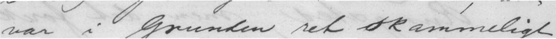var i Grunden ret skammeligt
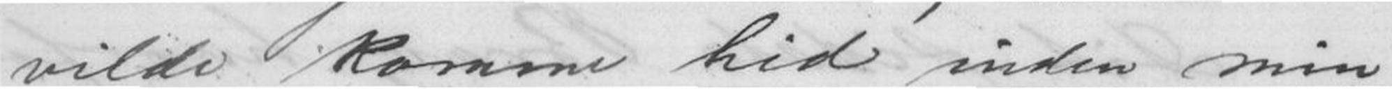vilde komme hid inden min
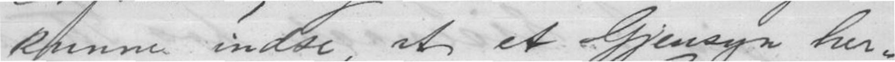kunne indse, at et Gjensyn her-
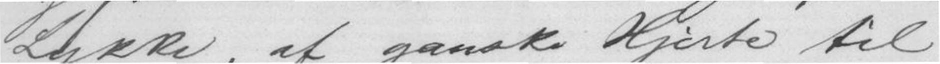Lykke, af ganske Hjerte til
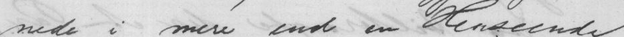nede i mere end en Henseende
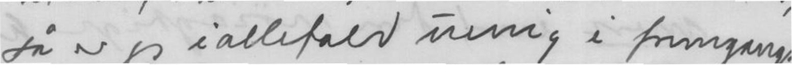så er jeg iallefald uenig i fremgangs-
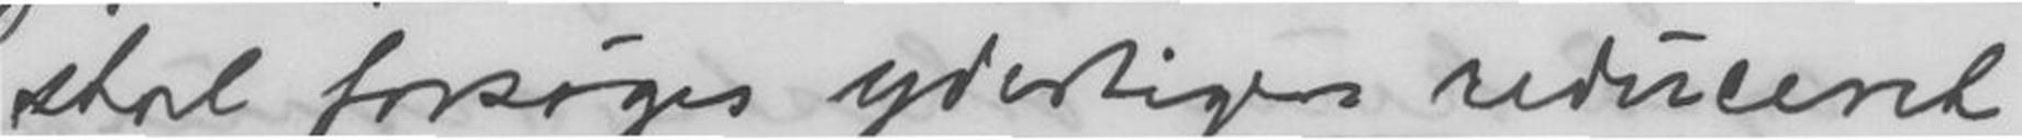skal forsøges yderligere reduceret
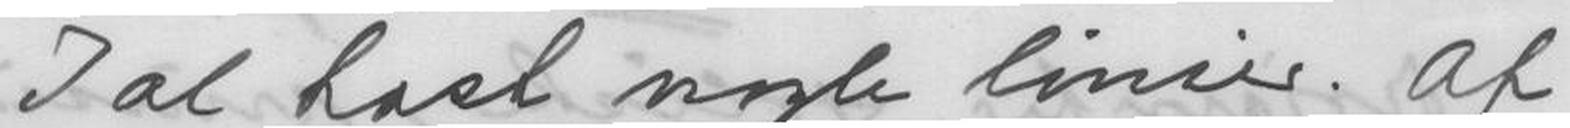I al hast nogle linier. Af
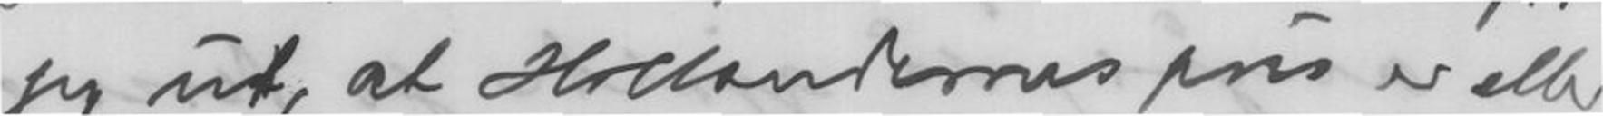jeg ut, at Hollændernes pris er eller
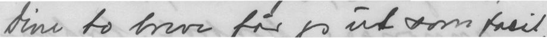dine to breve får jeg ud som fasit,
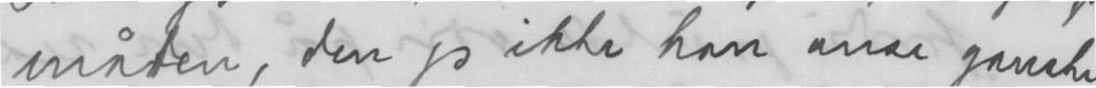måten, den jeg ikke kan anse ganske
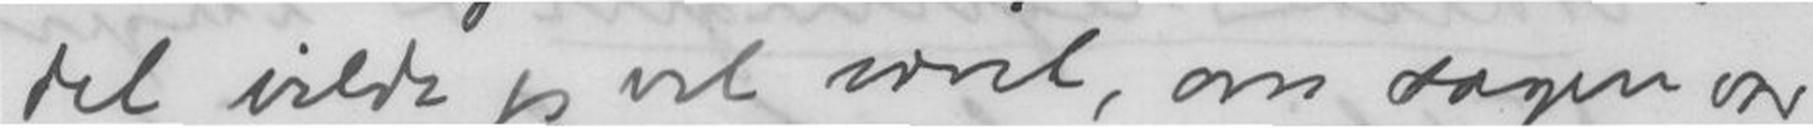det vilde jeg vel været, om sagen var
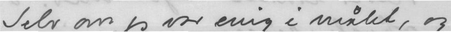Selv om jeg var enig i målet, og
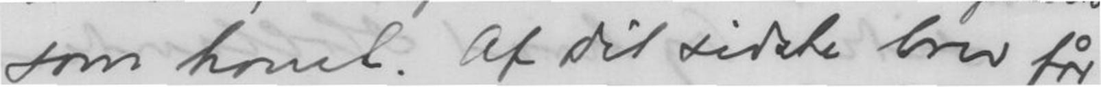som korect. Af dit sidste brev får
